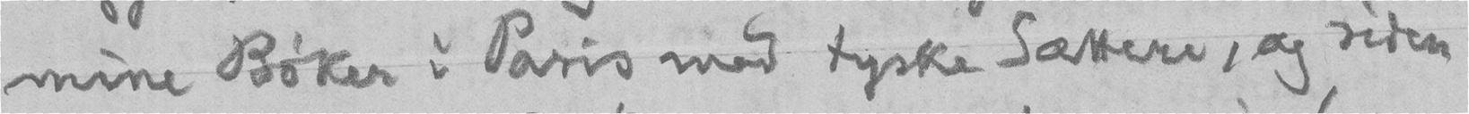mine Bøker i Paris med tyske Sættere, og siden
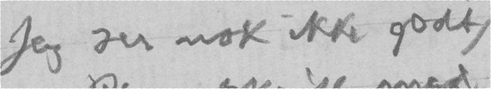Jeg ser nok ikke godt
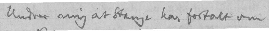Undrer mig at Stange har fortalt om
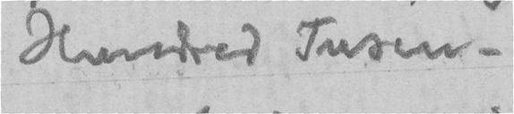Hundred Tusen.
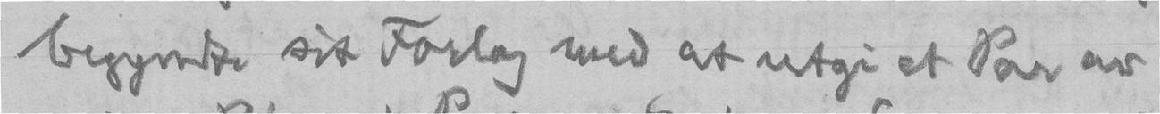begyndte sit Forlag med at utgi et Par av
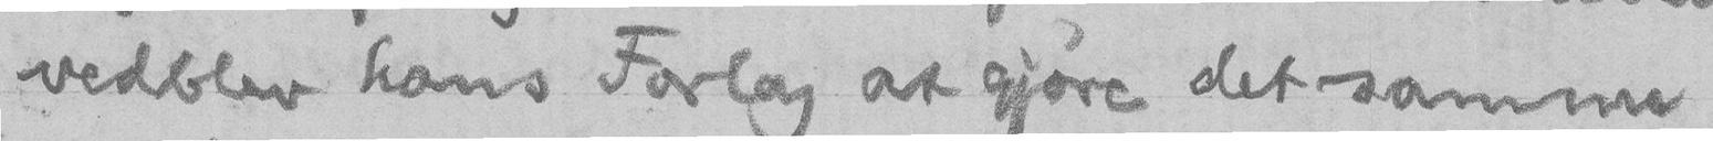vedblev hans Forlag at gjøre det samme
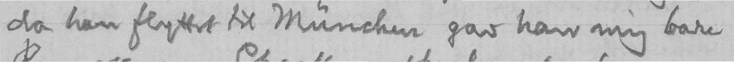da han flyttet til München gav han mig bare
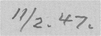11/2. 47.
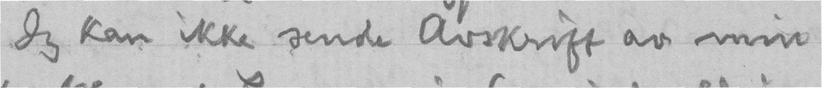Jeg kan ikke sende Avskrift av min
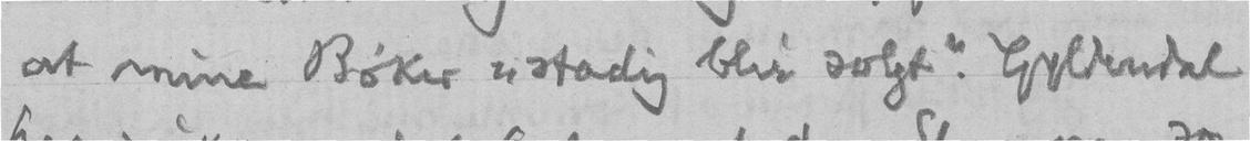at mine Bøker "stadig blir solgt". Gyldendal
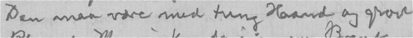Den maa være med tung Haand og grov
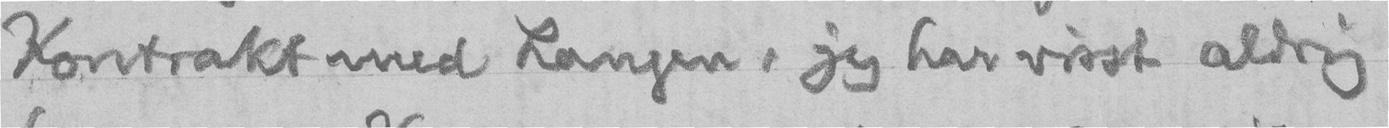Kontrakt med Langen, jeg har visst aldrig
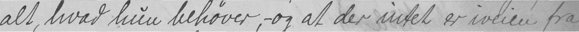alt, hvad hun behøver, - og at der intet er iveien fra
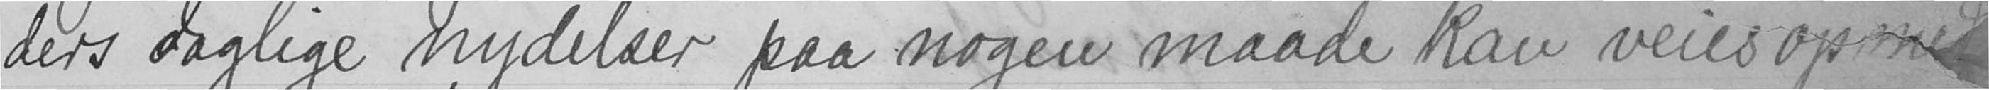ders daglige nydelser paa nogen maade kan veies op med
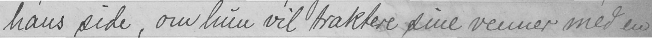hans side om hun vil traktere sine venner med en
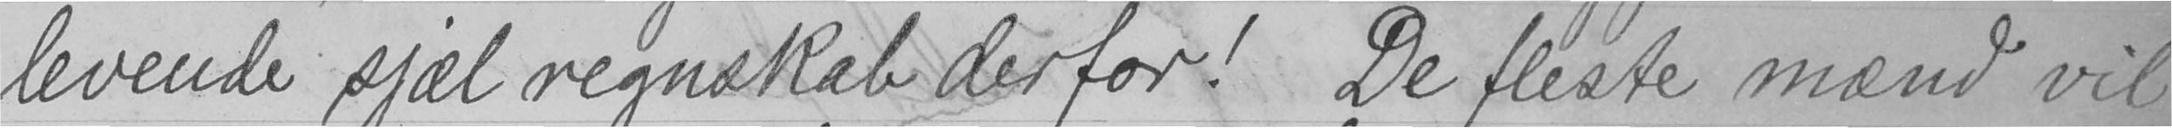levende sjæl regnskab derfor! De fleste mænd vil
